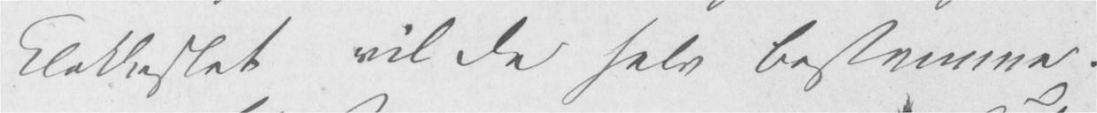Klokkeslet vil De selv bestemme.
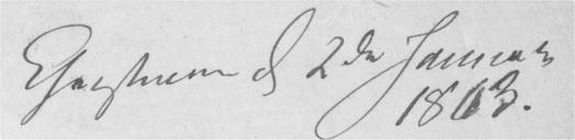Christiania d 2den Januar
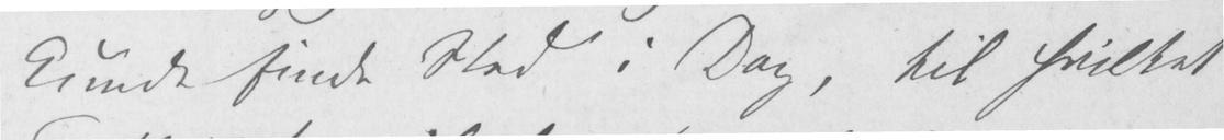kunde finde Sted i Dag, til hvilket
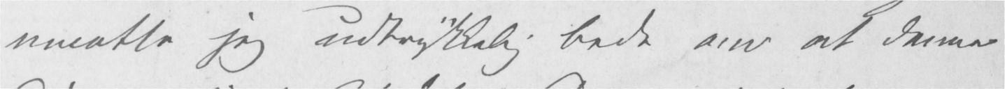maatte jeg udtrykkelig bede om at denne
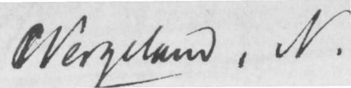Wergeland, N.
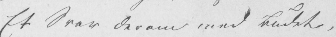Et Svar derom med Budet,
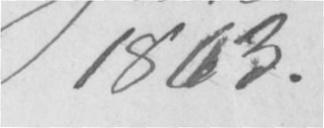1863.
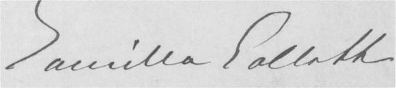Camilla Collett
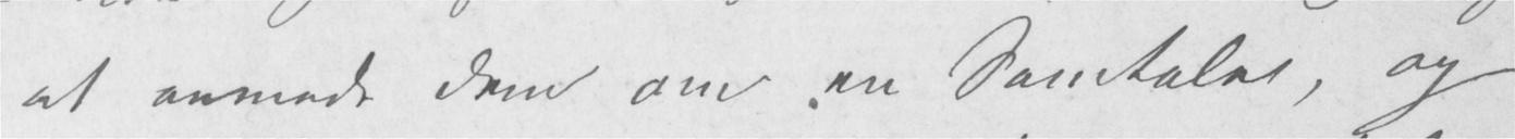at anmode Dem om en Samtale, og
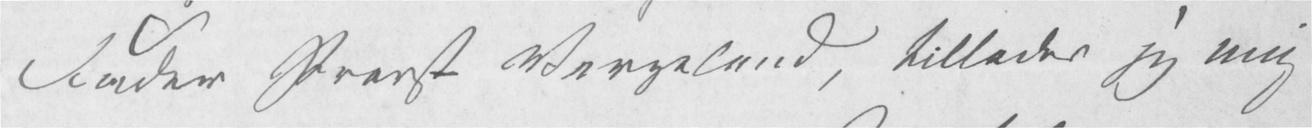Fader Provst Wergeland, tillader jeg mig
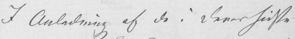I Anledning af de i Deres sidste
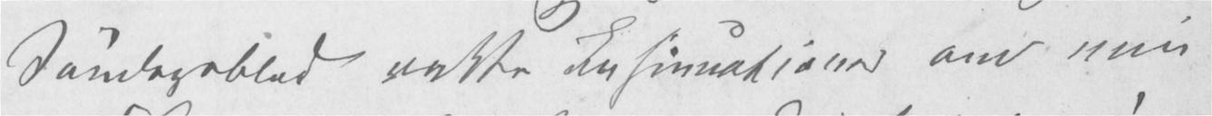Søndagsblad vakte Insinuationer om min
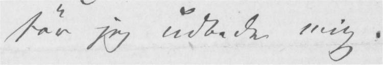tør jeg udbede mig.
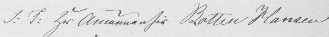S: T: Hr Amanuensis Botten Hansen
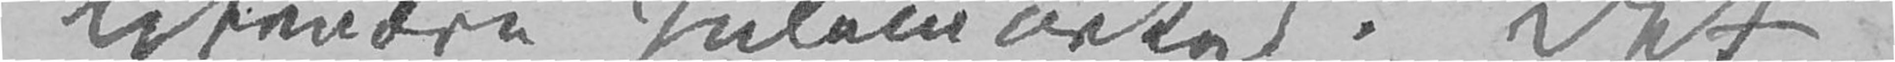literære Julemarked. Det
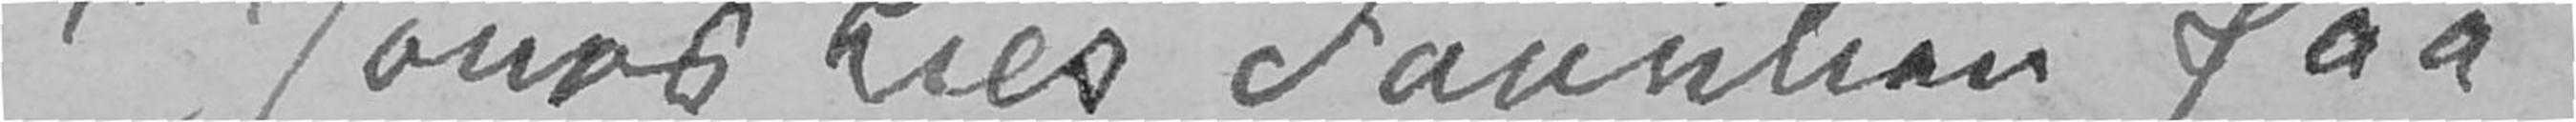«Jonas Lies «Familien paa
